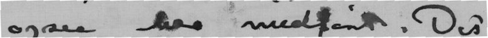ogsaa har medført. Dit
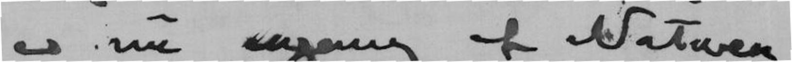er nu engang af Naturen
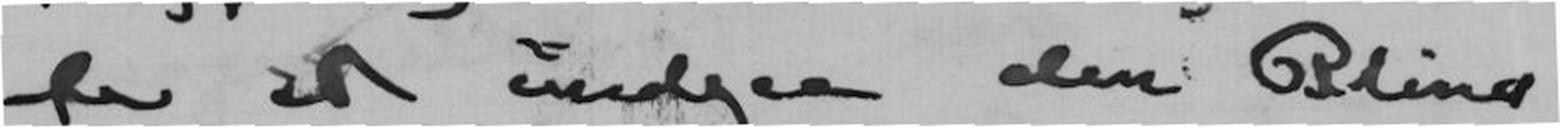for at undgaa den Blind-
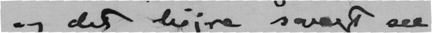og det højre svagt see
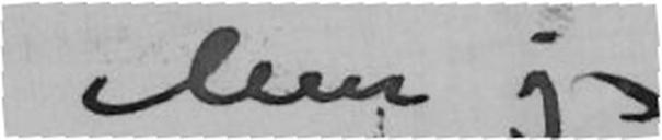Men jeg
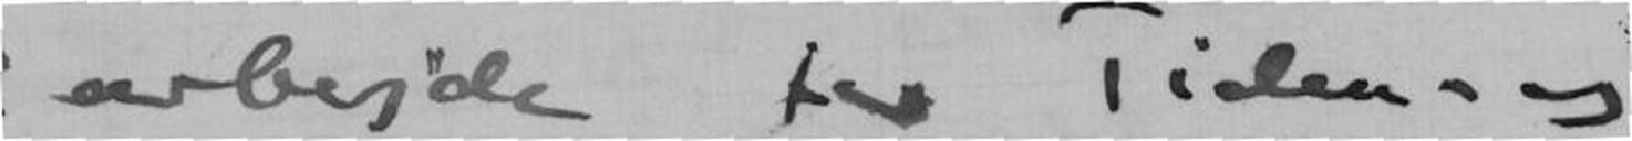arbejde for Tiden og
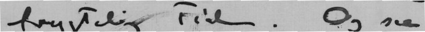og frygtelig tid. Og saa
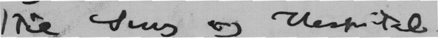til Seng og Hospital
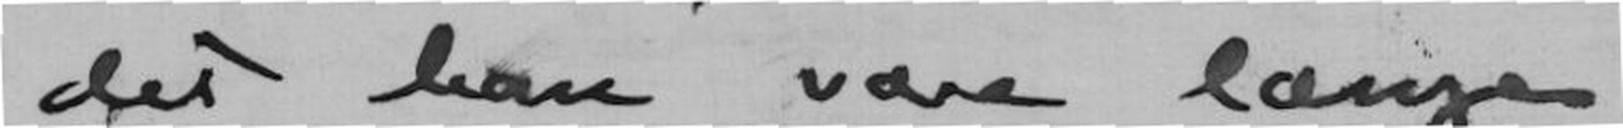det kan vare længe
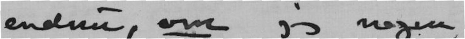endnu, om jeg nogen-
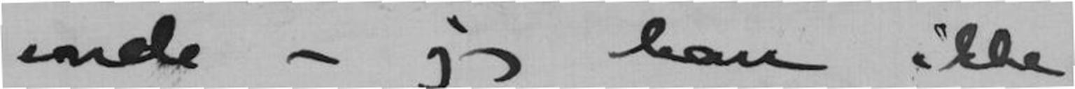enda - jeg kan ikke
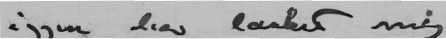igjen har lænket mig
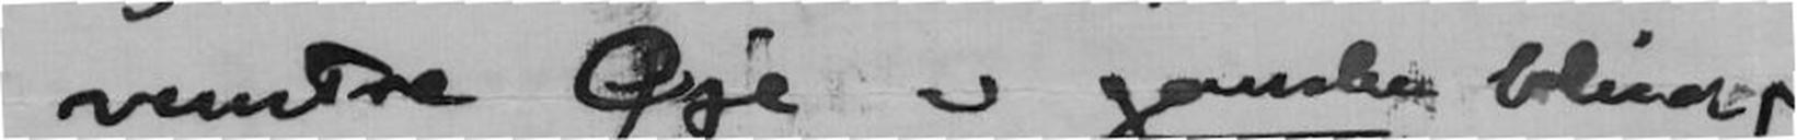venstre Øje er ganske blindet
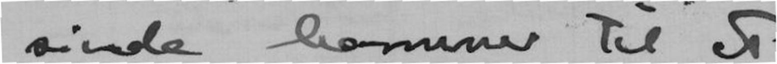sinde kommer til at
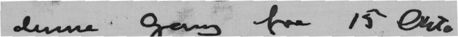denne Gang fra 15 Okto-
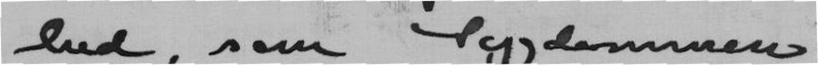hed, som Sygdommen
